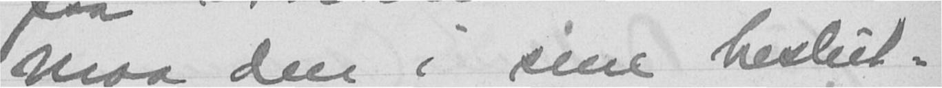maa den i sine beslut-
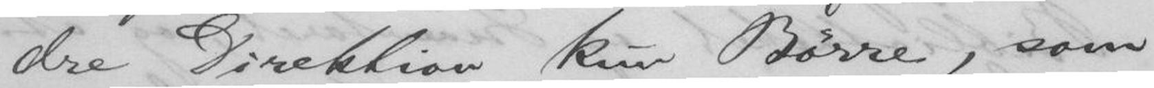dre Direktion kun Børre, som
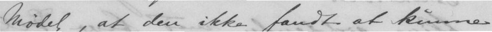Mødet, at den ikke fandt at kunne
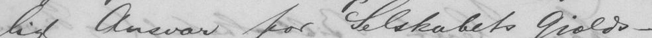ligt Ansvar for Selskabets Gjælds-
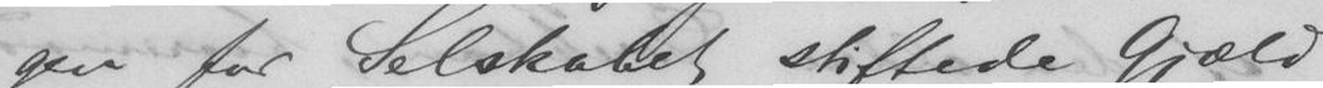gen for Selskabet stiftede Gjæld
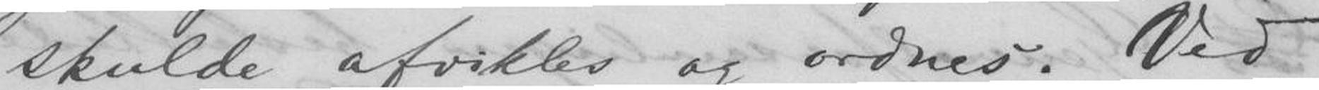skulde afvikles og ordnes. Ved
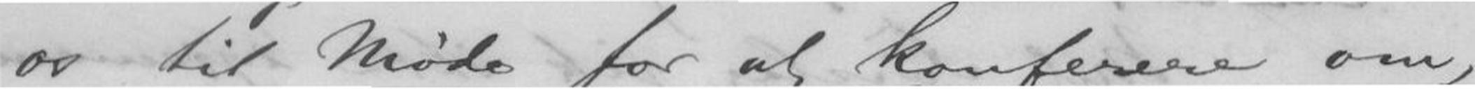os til Møde for at konferere om,
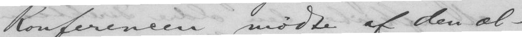Konferencen mødte af den æl-
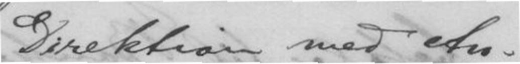Direktion med An-
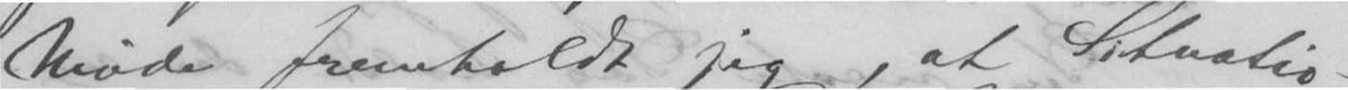Møde fremholdt jeg, at Situatio-
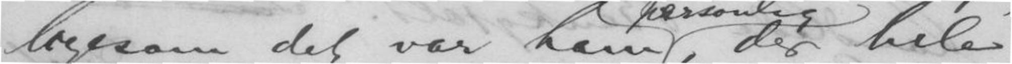ligesom det var ham, der hele
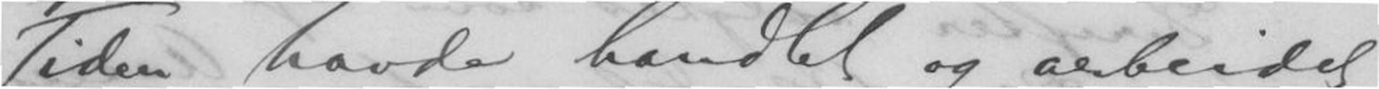Tiden havde handlet og arbeidet
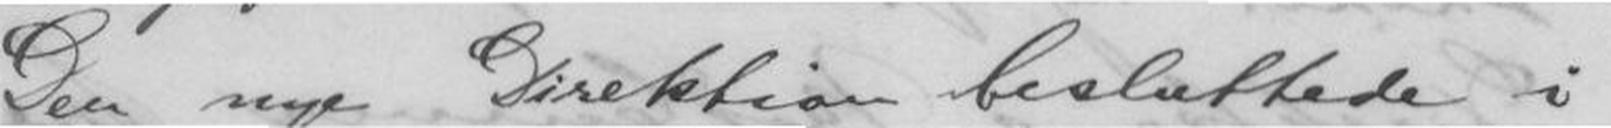Den nye Direktion besluttede i
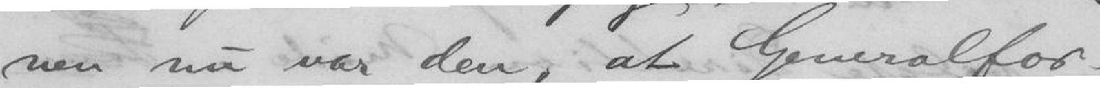nen nu var den, at Generalfor-
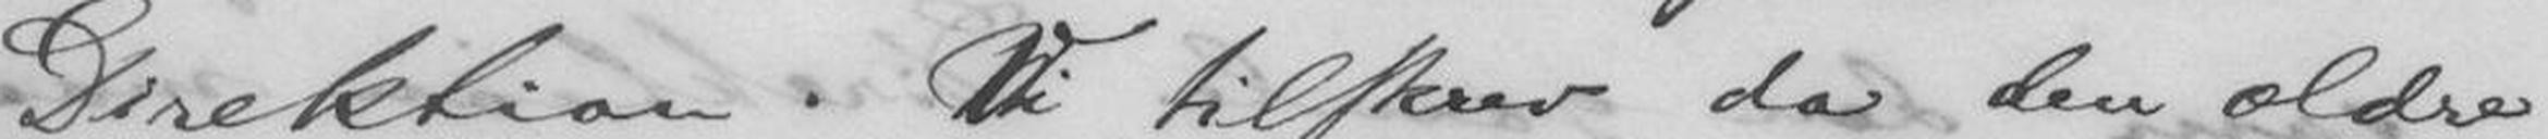Direktion. Vi tilskrev da den ældre
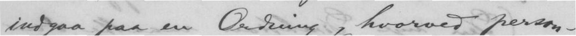indgaa paa en Ordning, hvorved person-
